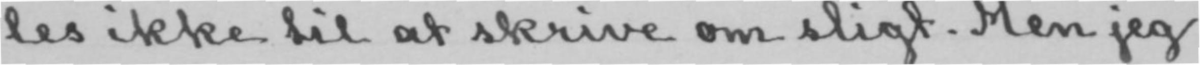les ikke til at skrive om sligt. Men jeg
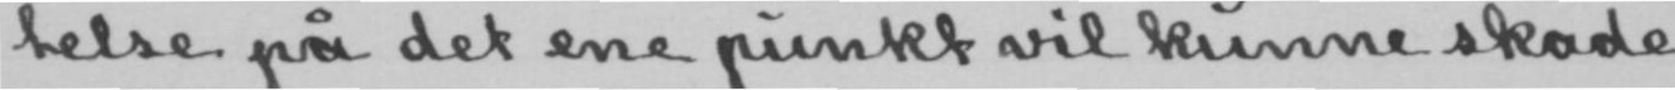telse på det ene punkt vil kunne skade
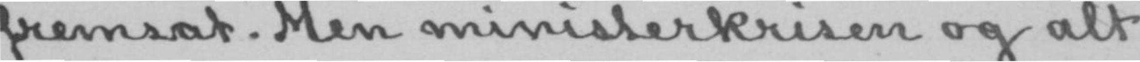fremsat. Men ministerkrisen og alt
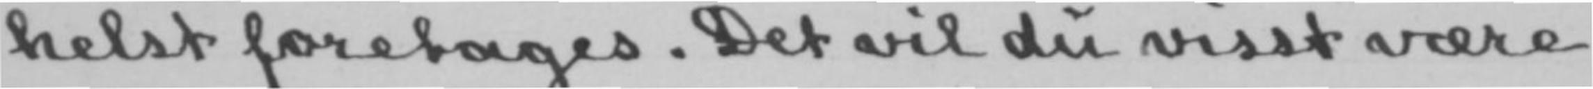helst foretages. Det vil du visst være
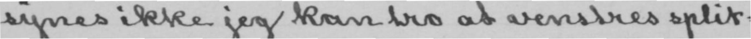synes ikke jeg kan tro at venstres split-
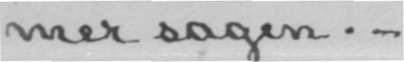mer sagen.-
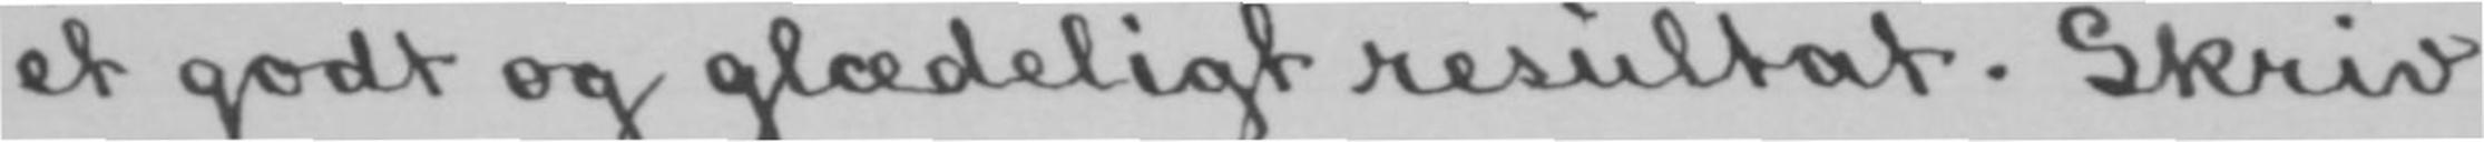et godt og glædeligt resultat. Skriv
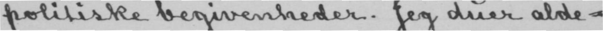politiske begivenheder. Jeg duer alde-
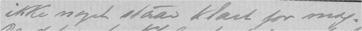ikke noget staar klart for mig.
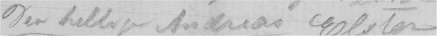Den hellige Andreas Elster
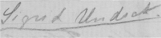Sigrid Undset.
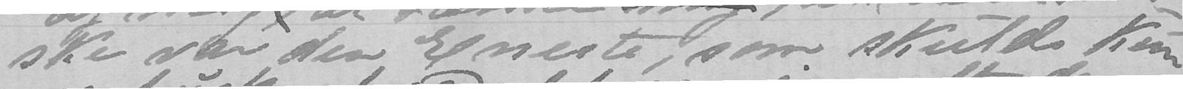ske var den Eneste, som skulde kun-
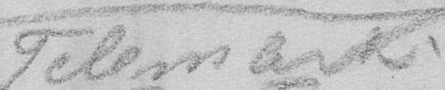Telemark.
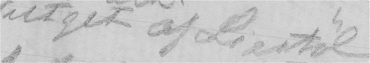utgit af Liestøl
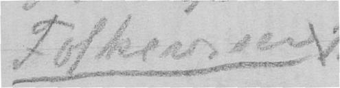Folkeviser
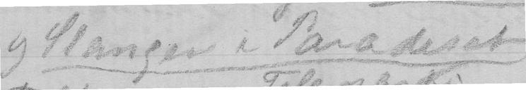og Slangen i Paradiset
